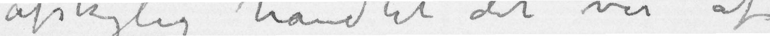avskyelig handlet det var af
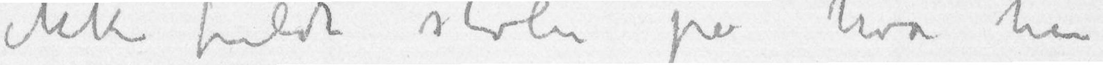ikke fuldt stoler på hva han
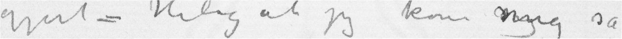gjort – Helig at jeg kom mig så
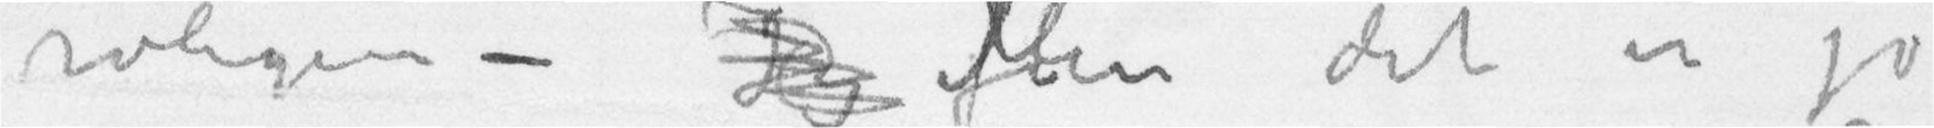roligere – Jeg Men det er jo
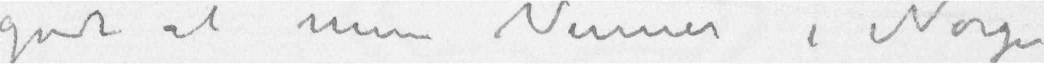godt at mine Venner i Norge
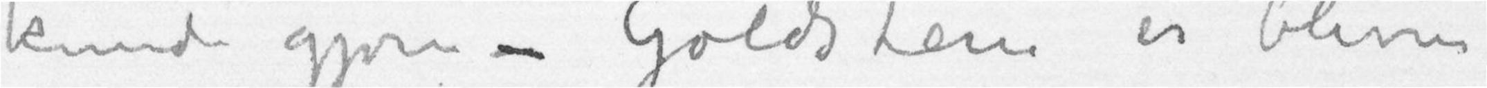kunde gjøre – Goldstein er bleven
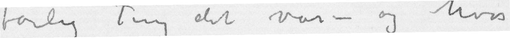farlig Ting det var – og hvor
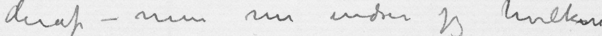deraf – men nu undrer jeg hvilken
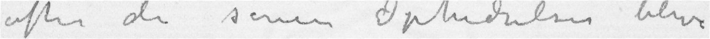efter de senere Ophidselser blive
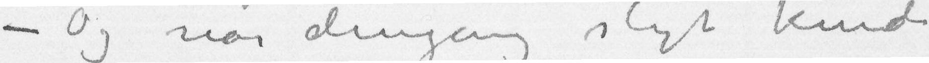– Og når dengang sligt kunde
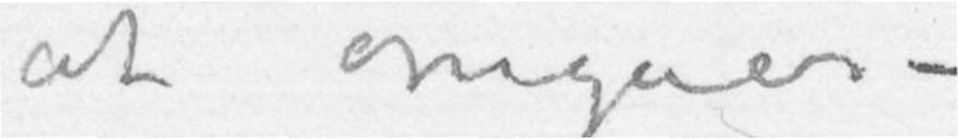at omgåes –
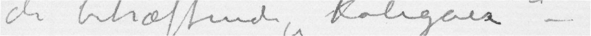de betræffende «Kolegaer» –
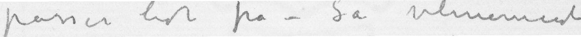passer lidt på – Så velmenende
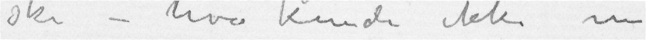ske – hva kunde ikke nu
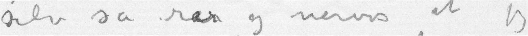selv så rar og nervøs at jeg
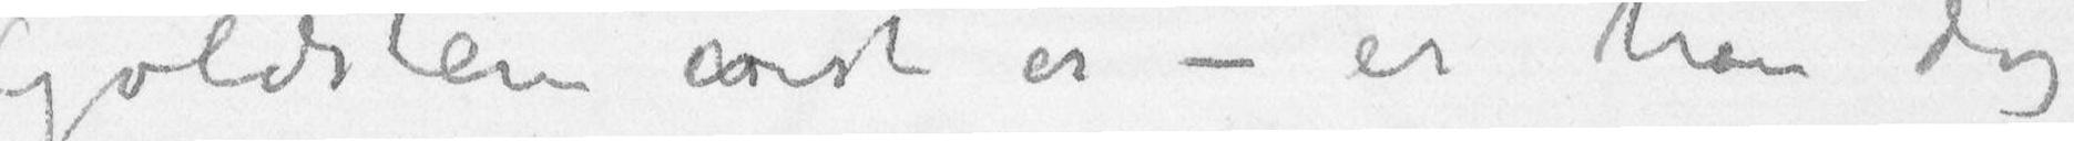Goldstein vist er – er han dog
